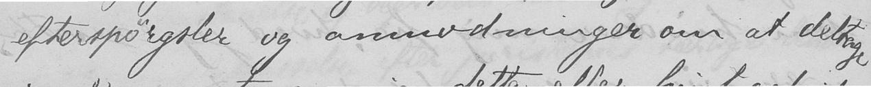efterspørgsler og anmodninger om at deltage
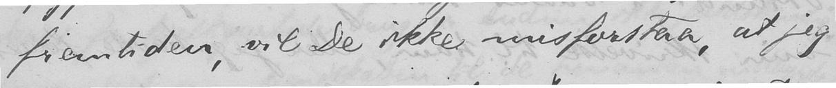fremtiden, vil De ikke misforstaa, at jeg
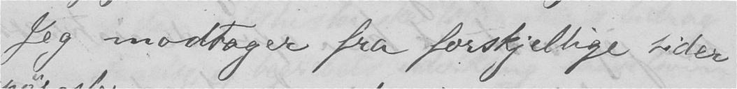Jeg modtager fra forskjellige sider
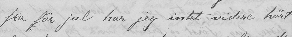fra før jul har jeg intet videre hørt
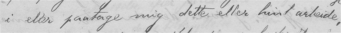i eller paatage mig dette eller hint arbeide,
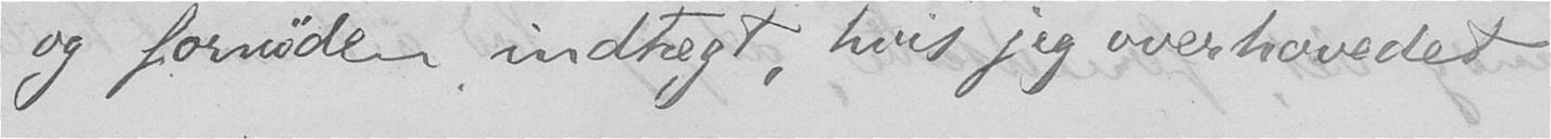og fornøden indtægt, hvis jeg overhovedet
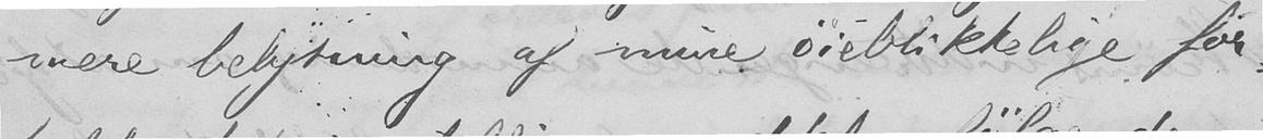mere belysning af mine øieblikkelige for-
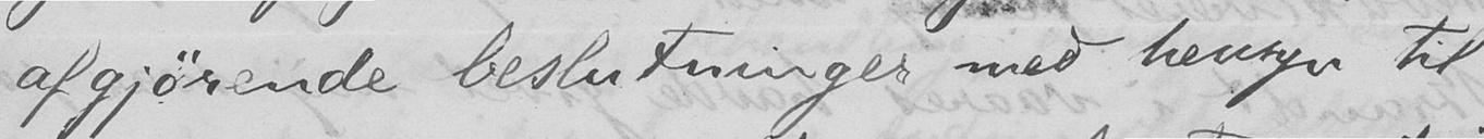afgjørende beslutninger med hensyn til
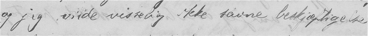og jeg vilde visselig ikke savne beskjæftigelse
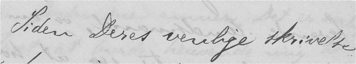Siden Deres venlige skrivelse
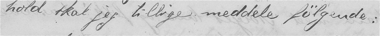hold skal jeg tillige meddele følgende:
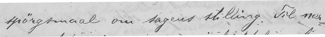spørgsmaal om sagens stilling. Til nær-
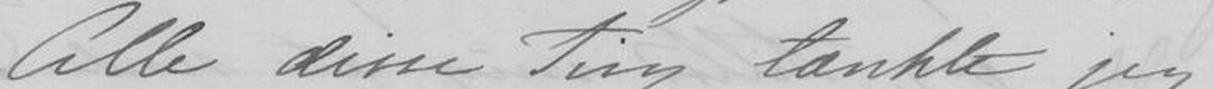Alle disse Ting tænkte jeg
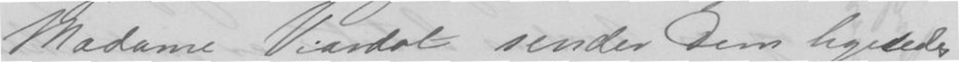Madame Viardot sender Dem ligeledes
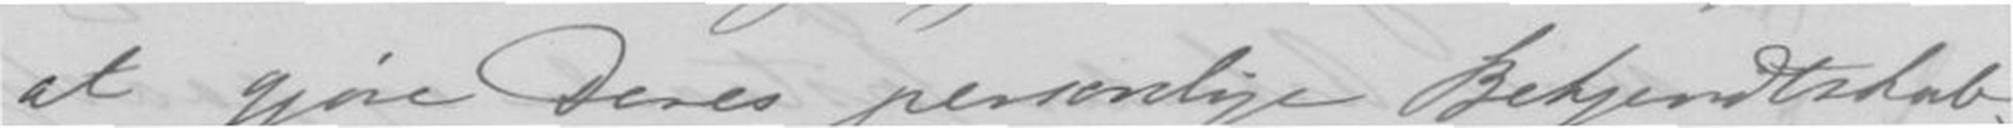at gjøre Deres personlige Bekjendtskab.
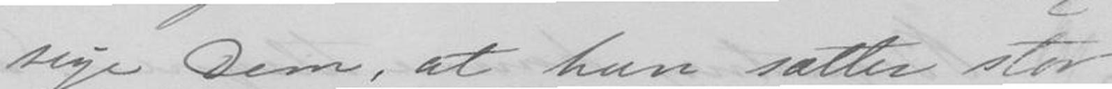sige Dem, at hun sætter stor
